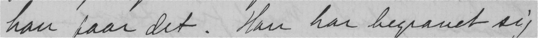han faar det. Han har begravet sig
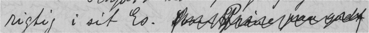rigtig i sit Es.
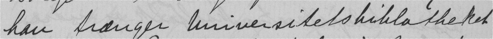han trænger Universitetsbiblotheket
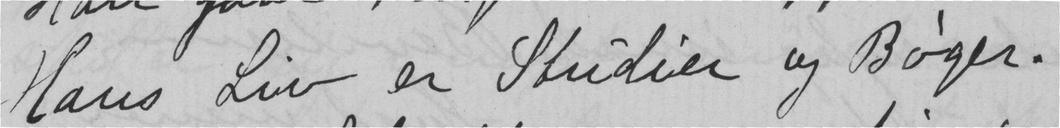Hans Liv er Studier og Bøger.
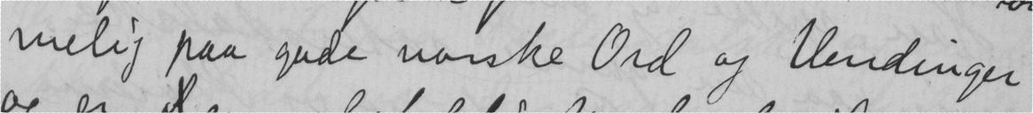melig paa gode norske Ord og vendinger
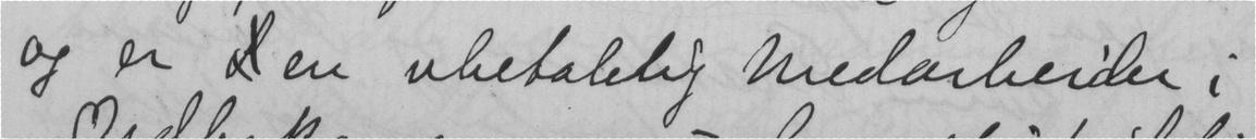og er Den ubetalelig medarbeider i
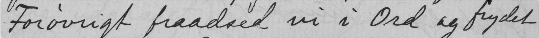Forøvrigt fraadsed vi i Ord og frydet
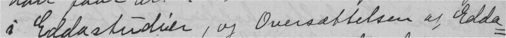i Eddastudier, og Oversættelsen af Edda-
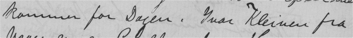kommer for Dagen. Ivar Kleiven fra
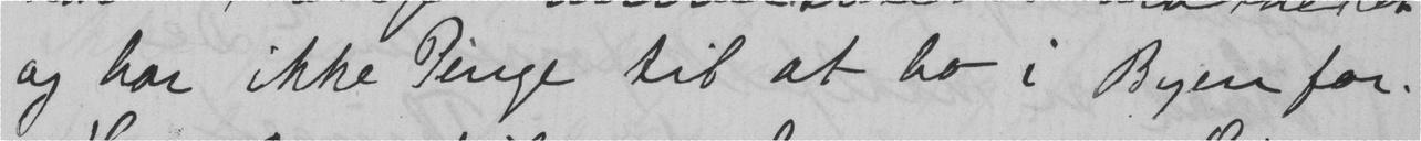og har ikke Penge til at bo i Byen for.
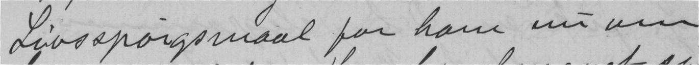Livsspørgsmaal for ham nu om
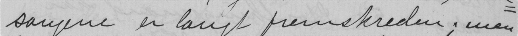sangene er langt fremskreden; men
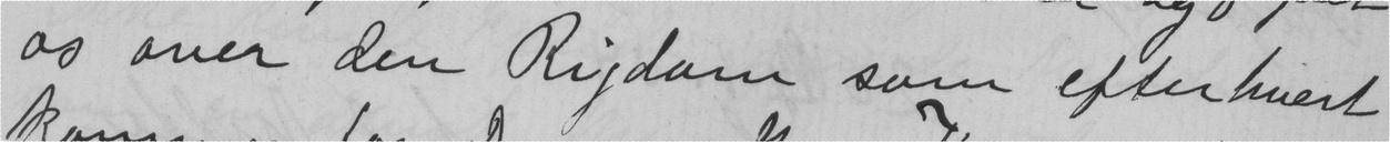os over den Rigdom som efterhvert
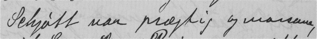Schjøtt var prægtig og morsom,
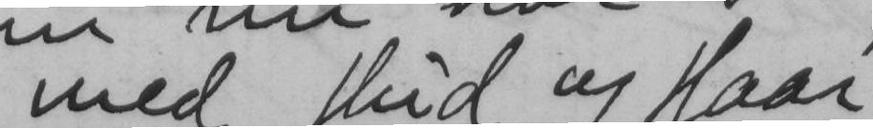med Hud og Haar
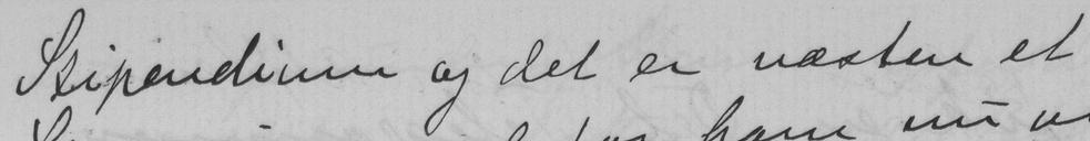Stipendium og det er næsten et
